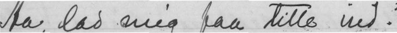Aa, lad mig faa titte ind!
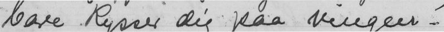bare Kysser dig paa vingen!
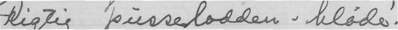Rigtig pusselodden - bløde!
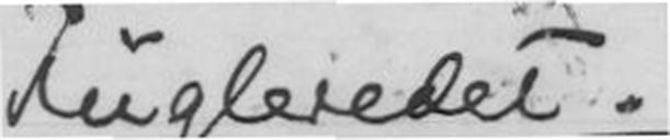Fugleredet.
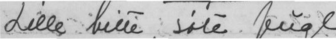Lille bitte, søte fugl
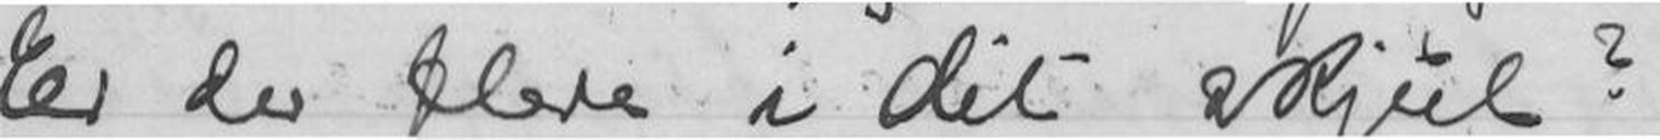Er der flere i dit skjul?
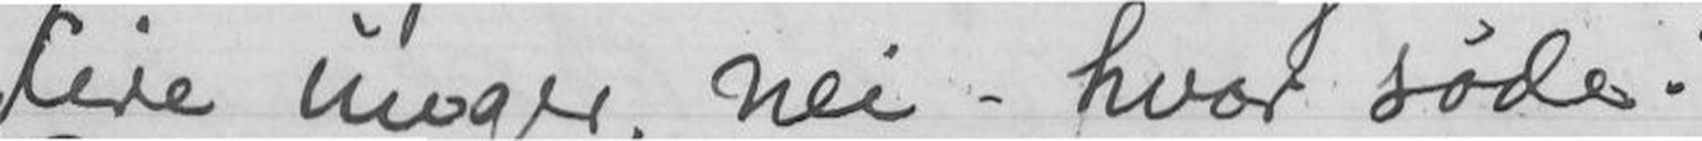Fire unger, nei - hvor søde!
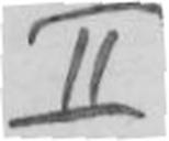II
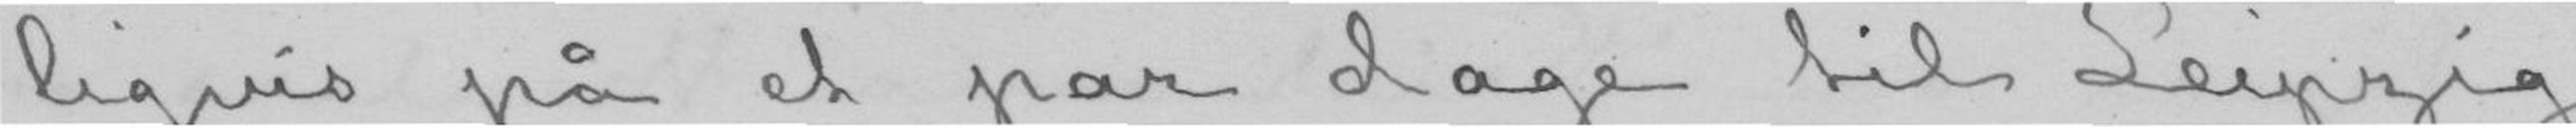ligvis på et par dage til Leipzig
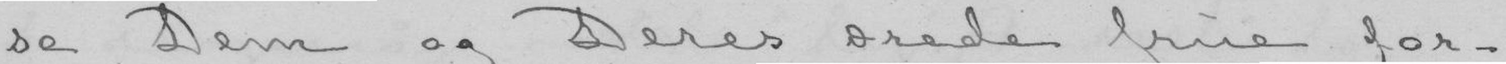se Dem og Deres ærede frue for-
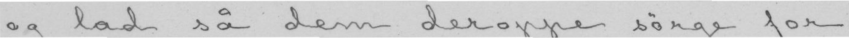og lad så dem deroppe sørge for
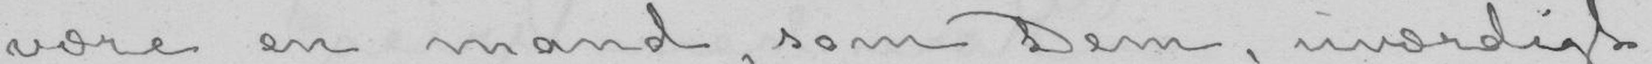være en mand, som Dem, uværdigt
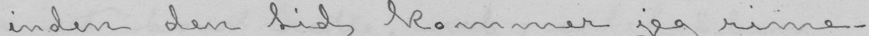inden den tid kommer jeg rime-
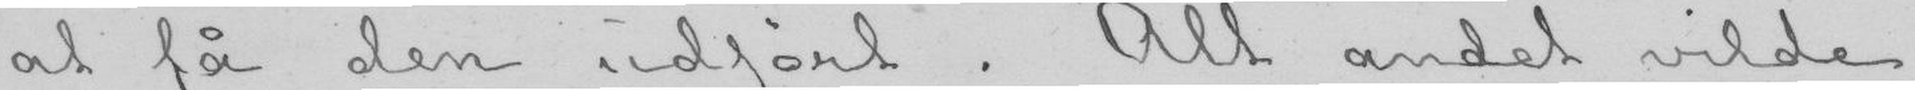at få den udført. Alt andet vilde
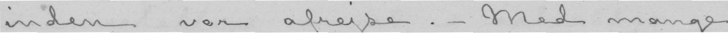inden vor afrejse. - Med mange
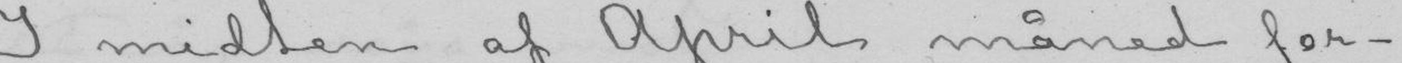I midten af April måned for-
